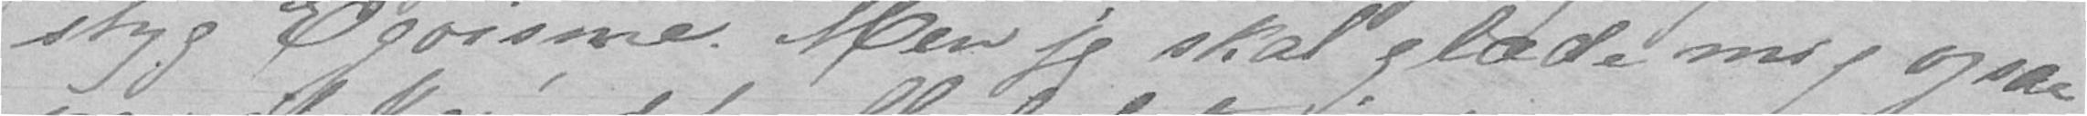styg Egoisme. Men jeg skal glæde mig ogsaa
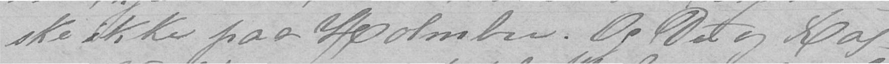ske ikke paa Holmbu. Og Du og Rag-
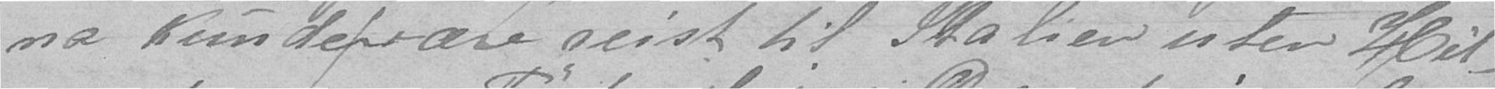na kunde være reist til Italien uten Hil-
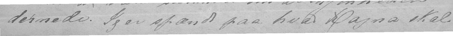dernede. Jeg er spændt paa hvad Ragna skal
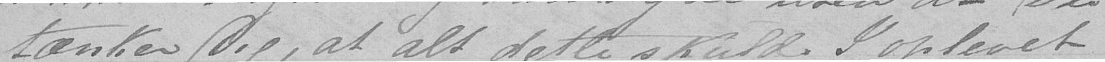tænker Dig, at alt dette skulde I oplevet
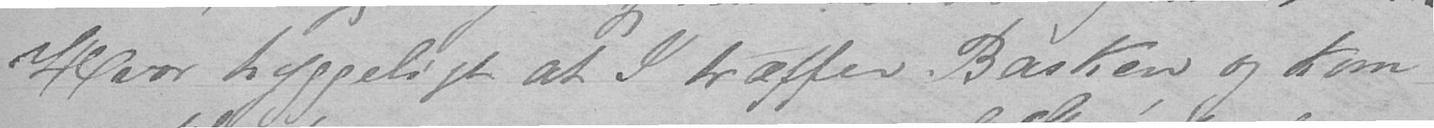Hvor hyggeligt at I træffer Basken og kom-
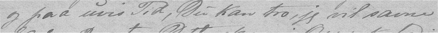og paa uvis Tid, Du kan tro, jeg vil savne
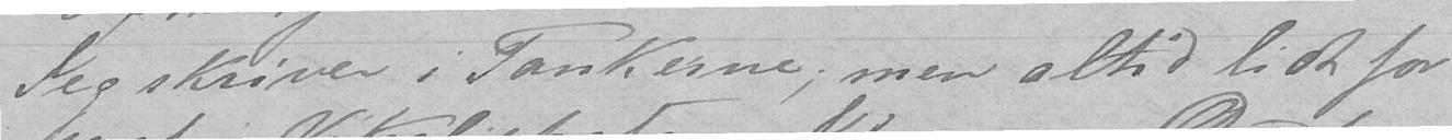Jeg skriver i Tankerne, men altid lidt for
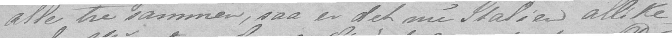alle tre sammen, saa er det nu Italien allike-
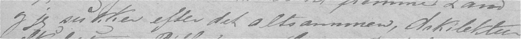og jeg sukker efter det altsammen, Arkitektur,
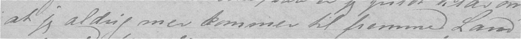at jeg aldrig mer kommer til fremmed Land
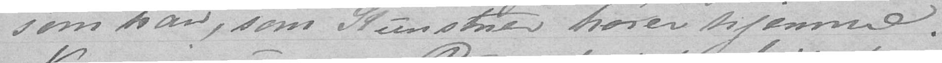som han, som Kunstner hører hjemme.
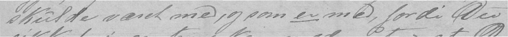skulde været med, og som er med, fordi Du
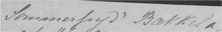Sommerfryd' Bækkela-
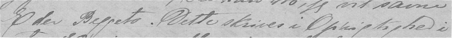Eder Beggeto. Dette skrives i Oprigtighed i
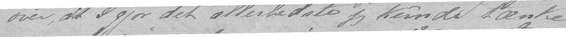over, at I gjør det allerbedste jeg kunde tænke
